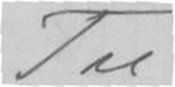Til
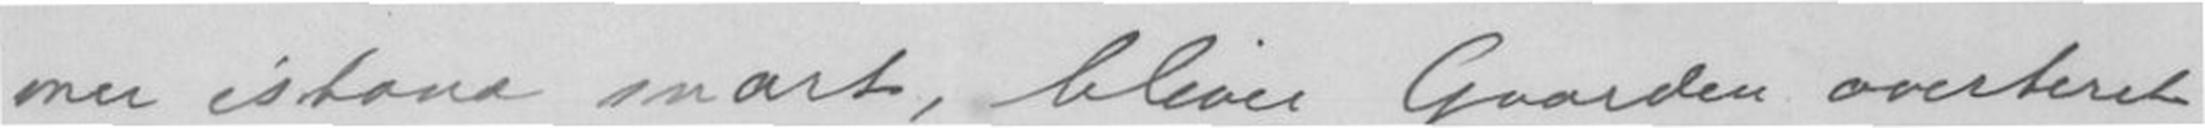mer istand snart, bliver Gaarden averteret
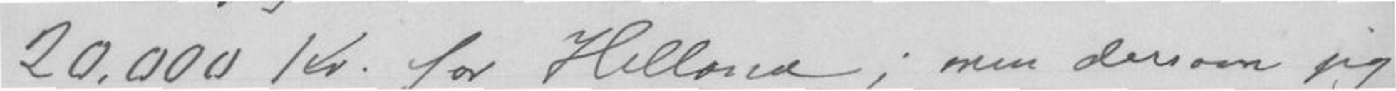20.000 Kr. for Helland; men dersom jeg
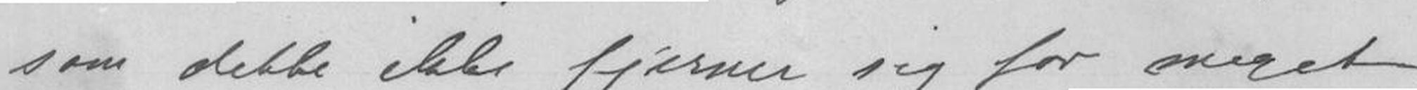som dette ikke fjerner sig for meget
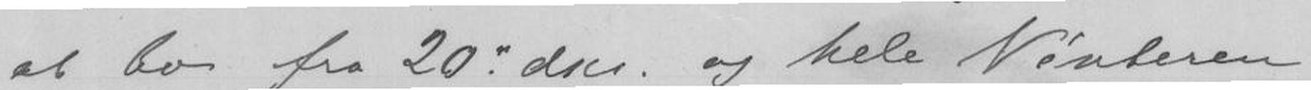at bo fra 20de dsc. og hele Vinteren.
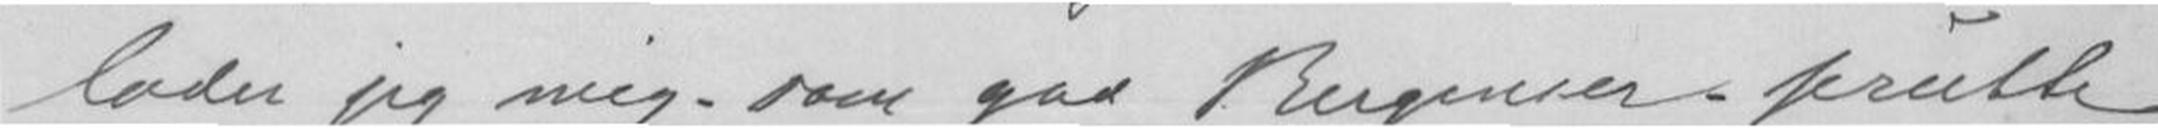lader jeg mig, som god Bergenser prutte.
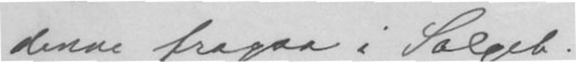denne fragaa i Salget.
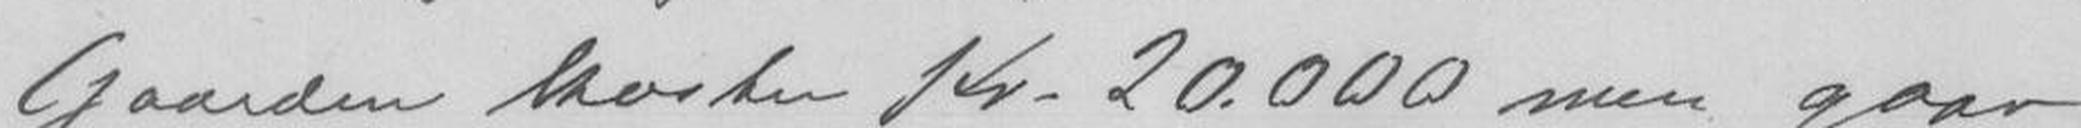Gaarden koster Kr. 20.000 men gaar
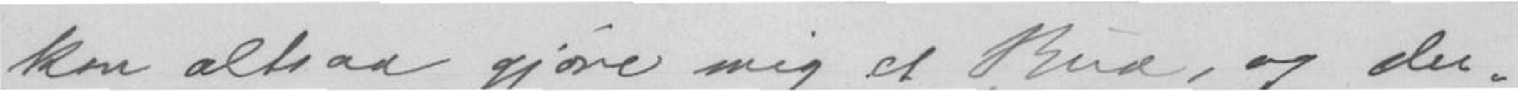kan altsaa gjøre mig et Bud, og der-
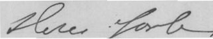Deres forb.
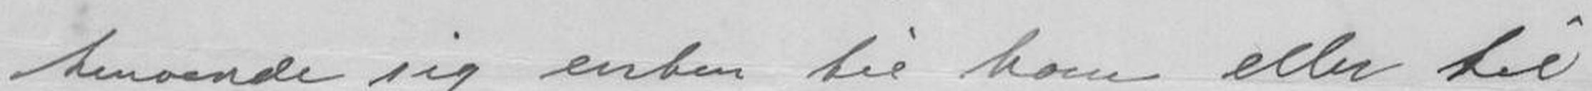henvende sig enten til han eller til
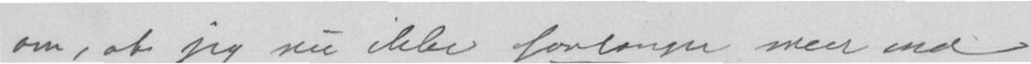om, at jeg nu ikke forlanger mere end
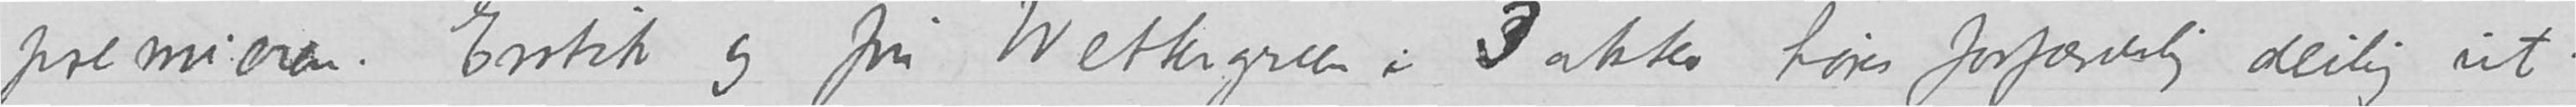premiæren. Erotik og fru Wettergreen i 3 akter høres forfærdelig deilig ut.
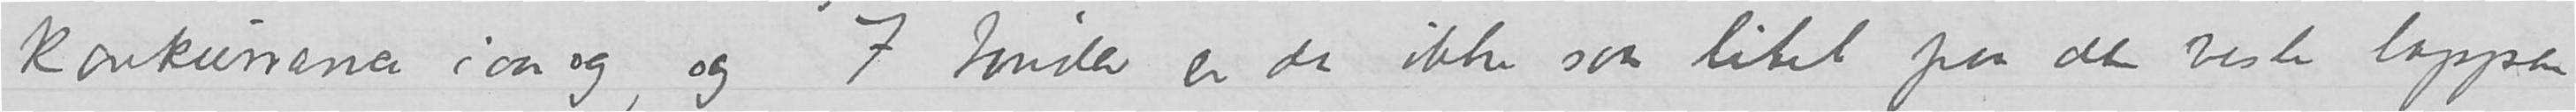konkurranse i aar og, og 7 tønder er da ikke saa litet paa de vesle lapper
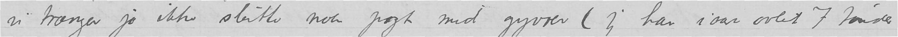vi trænger jo ikke slutte noen pagt med gyvoer (jeg har i aar avlet 7 tønder
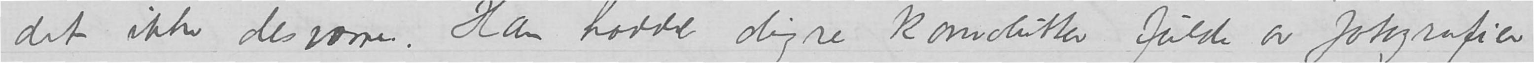det ikke desværre. Han hadde digre konvolutter fulde av fotografier
